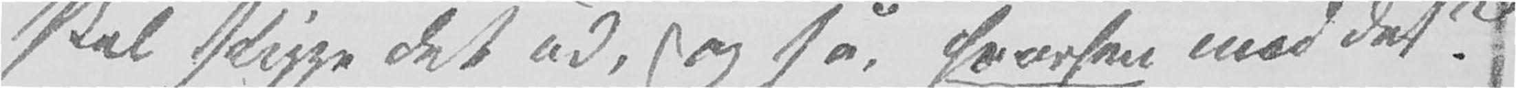skal slippe det ud, og så, hvorhen med det?
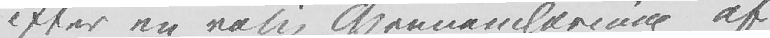efter en rolig Gjennemlæsning af
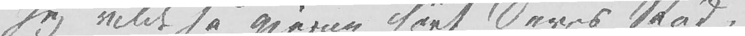Jeg vilde så gjerne hørt Deres Råd,
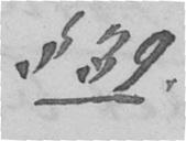§ 39.
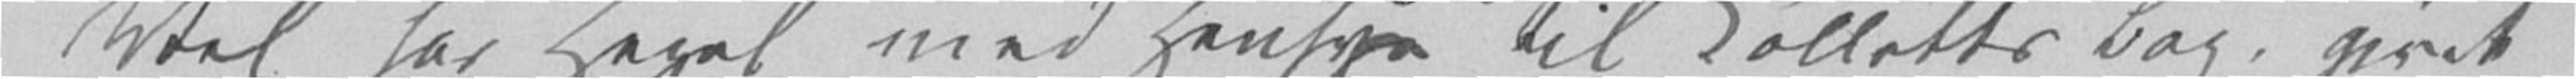Vel har Hegel med Hensyn til Colletts Bog, givet
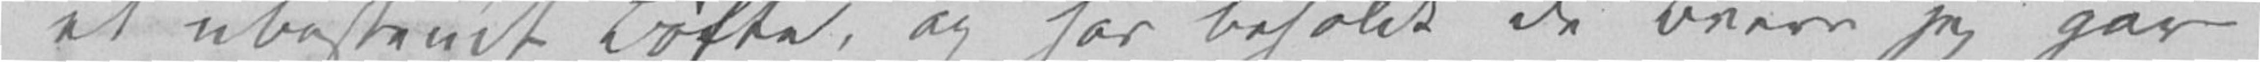et ubestemt Løfte, og har beholdt de Breve jeg gav
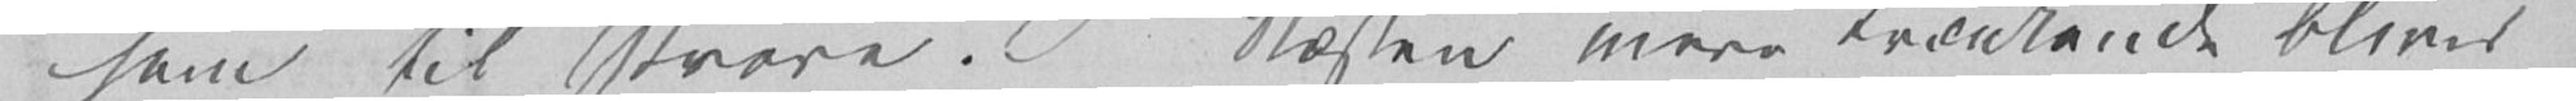ham til Prøve. Næsten mere krænkende bliver
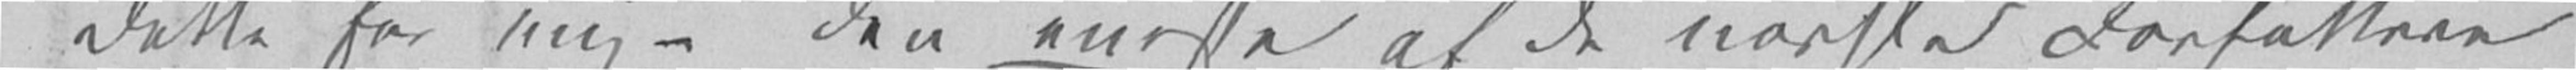dette for mig – den eneste af de norske Forfattere
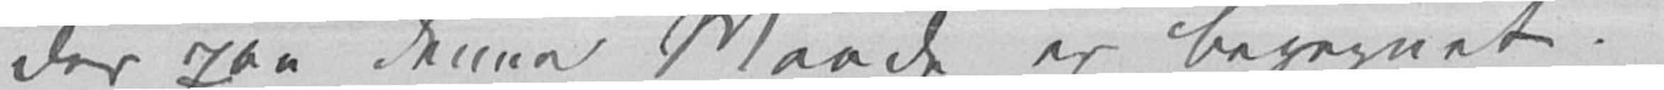der paa denne Maade er begegnet.
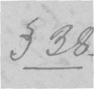§ 38.
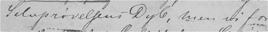Selvprøvelsens Dyb, men vi
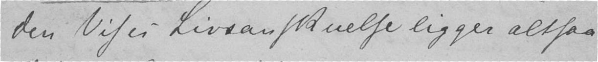den Vises Livsanskuelse ligger altsaa
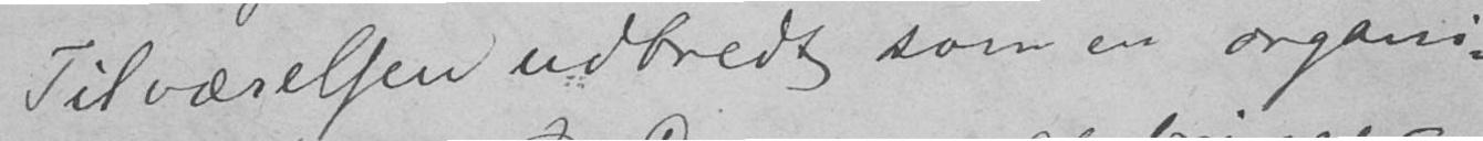Tilværelsen udbredt som en organi-
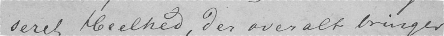seret Heelhed, der overalt bringer
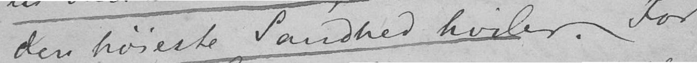den høieste Sandhed hviler. For
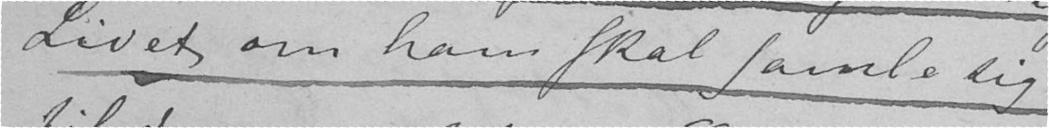Livet om ham skal samle sig
